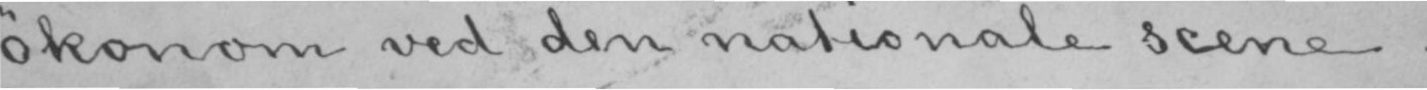økonom ved den nationale scene.
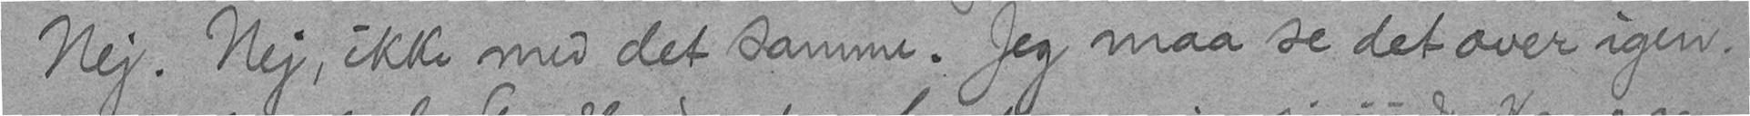Nej. Nej, ikke med det samme. Jeg maa se det over igen.
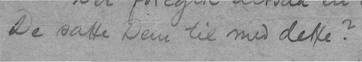De satte Dem til med dette?
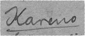Kareno
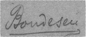Bondesen
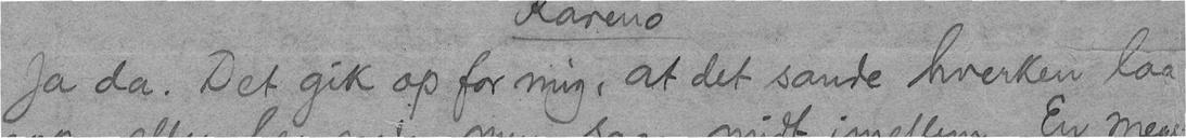Ja da. Det gik op for mig, at det sande hverken laa
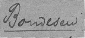Bondesen
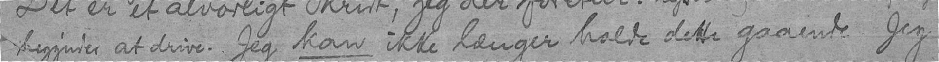begynder at drive. Jeg kan ikke længer holde dette gaaende Jeg
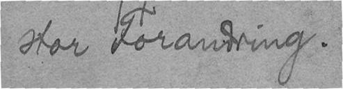stor Forandring.
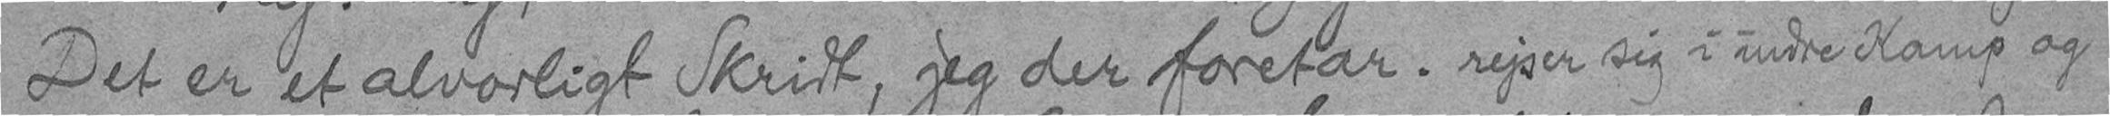Det er et alvorligt Skridt, jeg der foretar. rejser sig i indre Kamp og
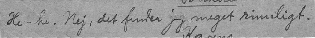He-he. Nej, det finder jeg meget rimeligt.
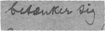betænker sig
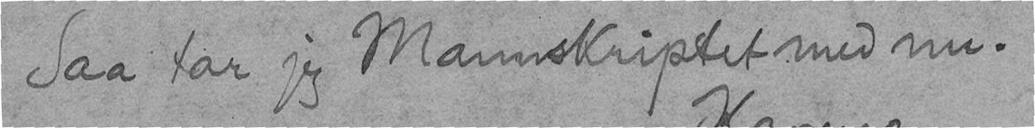Saa tar jeg Manuskriptet med nu.
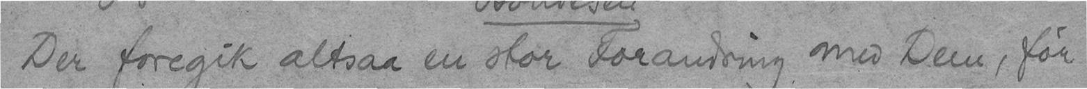Der foregik altsaa en stor Forandring med Dem, før
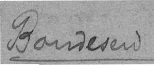Bondesen
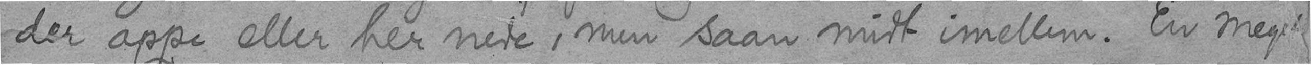der oppe eller her nede, men saan midt imellem. En meget
